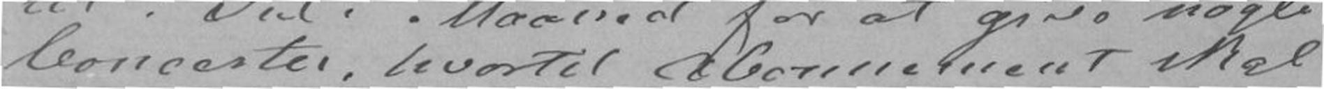Concerter, hvortil Abonnement skal
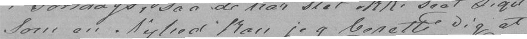Som en Nyhed kan jeg berette Dig at
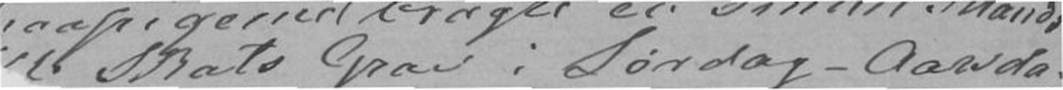Skats Grav i Lørdag - Aarsda-
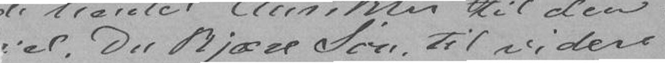el Du kjære Søn til videre
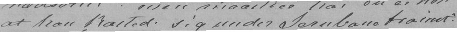at han kasted sig under Jernbanetrainer
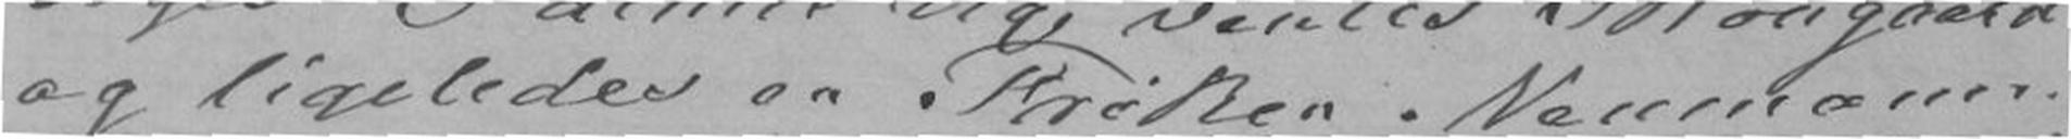og ligeledes en Frøken Neumann.
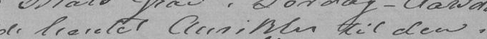d hentet Aurikler til den.
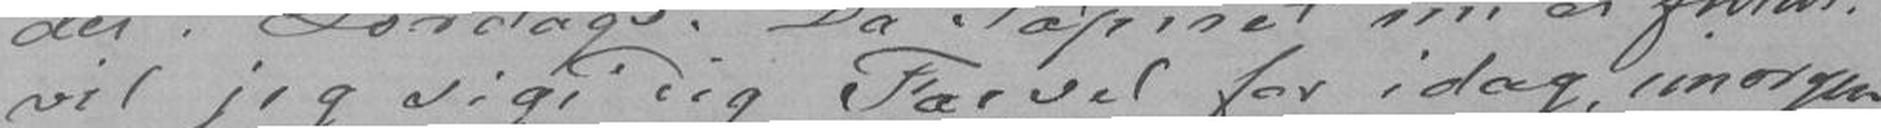vil jeg sige Dig Farvel for idag imorgen
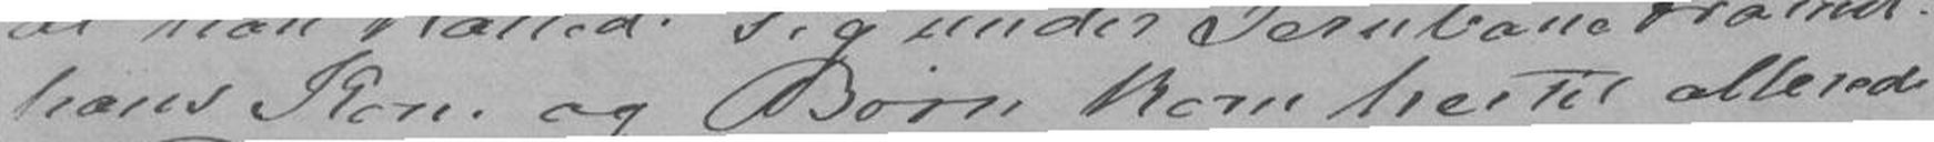hans Kone og Børn kom hertil allerede
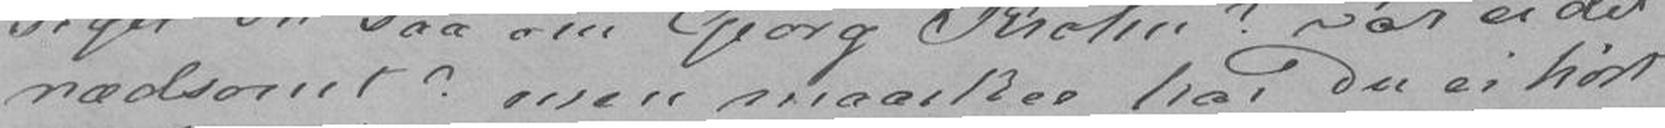rædsomt? men maaskee har Du ei hørt
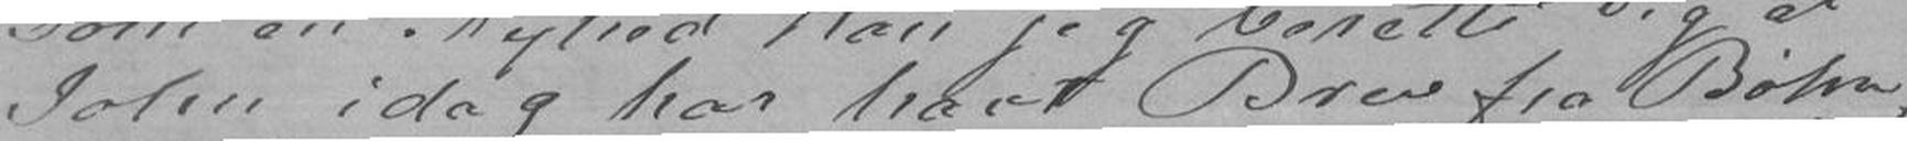John idag har havt Brev fra Bøhn,
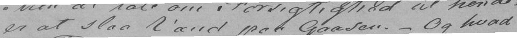er at slaa Vand paa Gaasen. - Og hvad
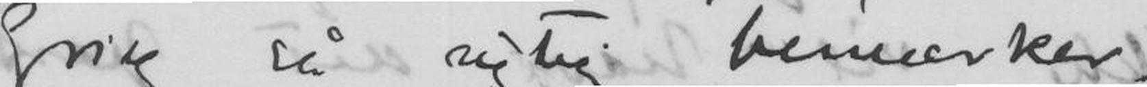Grieg så rigtig bemærker.
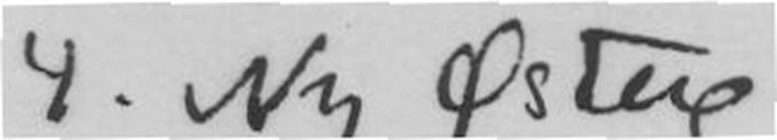4 Ny Østerg.
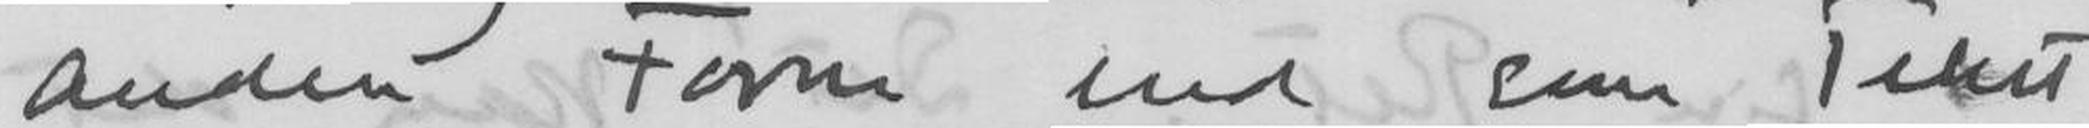anden Form end som Tekst
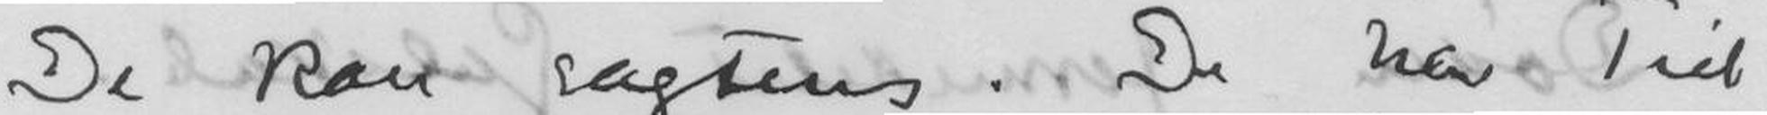De kan sagtens. De har Tid
